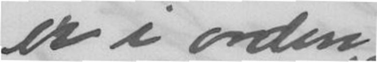er i orden.
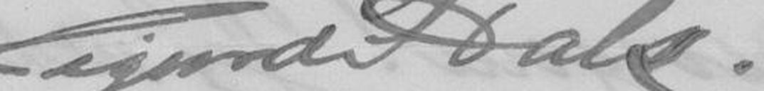Sigurd Hals.
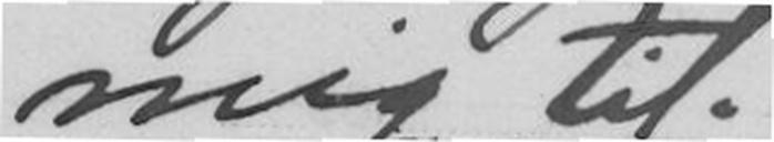mig til.
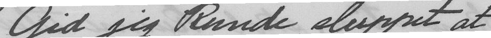Gid jeg kunde sluppet at
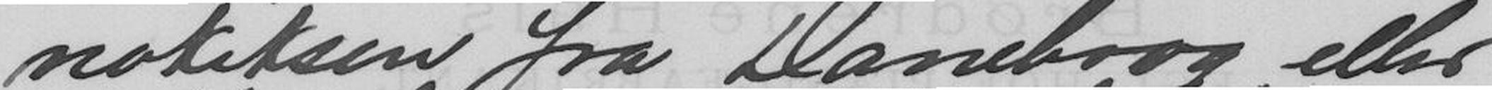notitsen fra Daneborg eller
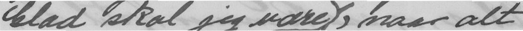Glad skal jeg være, naar alt
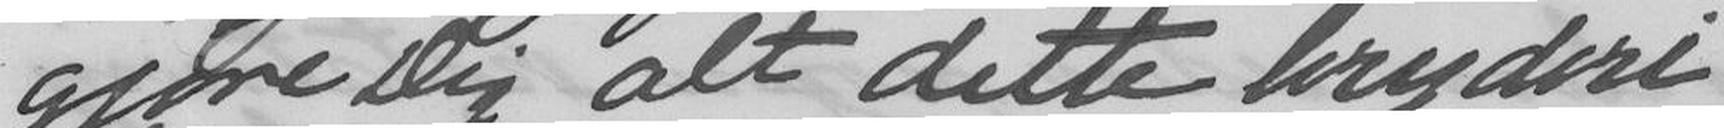gjøre alt dette bryderi.
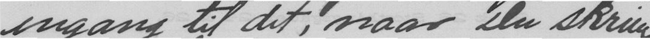engang til det, naar Du skriver
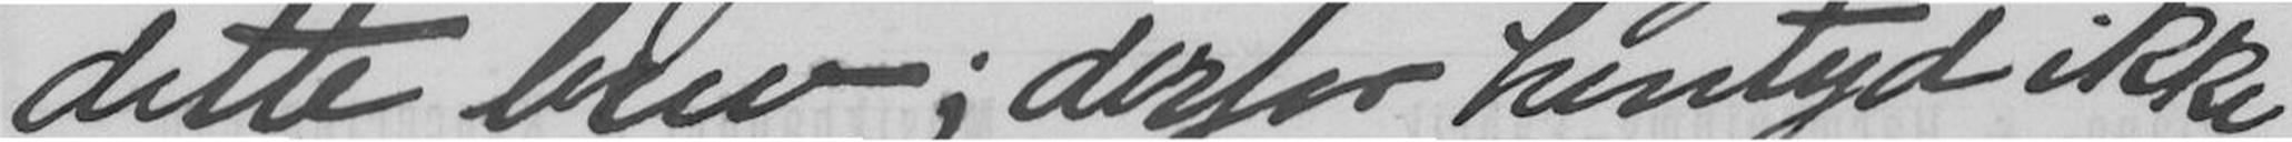dette brev; derfor hentyd ikke
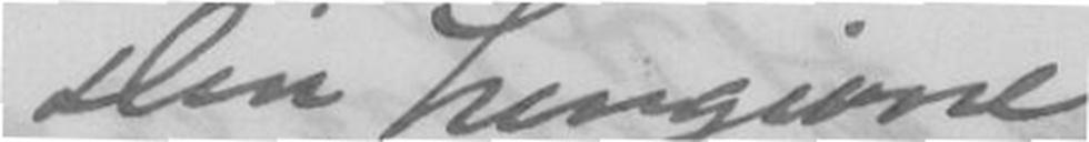Din hengivne
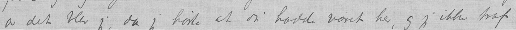av det blev jeg, da jeg hørte at du hadde været her, og jeg ikke traf
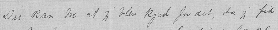Du kan tro at jeg blev kjed for det, da jeg fik
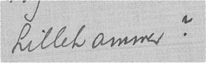Lillehammer?
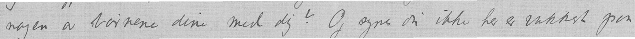nogen av børnene dine med dig? Og syns du ikke her er vakkert paa
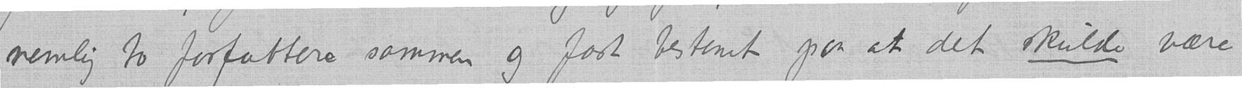nemlig to forfattere sammen og fast bestemt paa at det skulde være
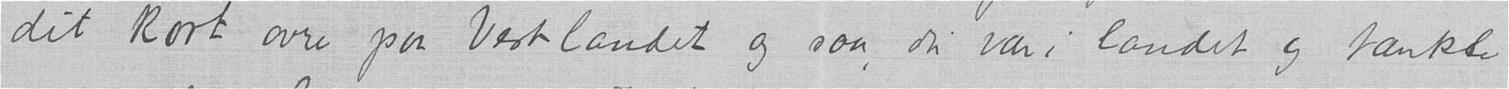dit kort over paa Vestlandet og saa, du var i landet og tænkte
